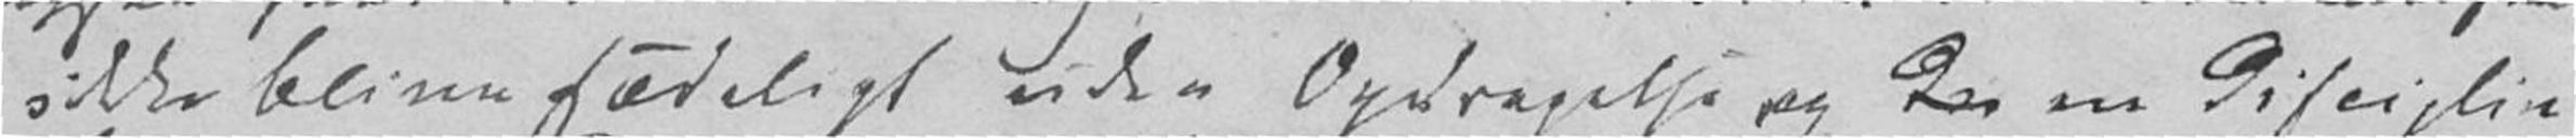ikke blive sædeligt uden Opdragelse og Der en Disciplin
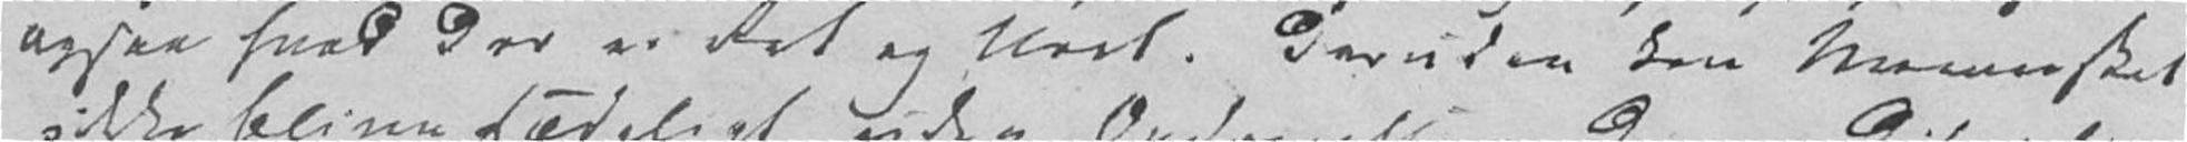ogsaa hvad der er Ret og Uret. Desuden kan Mennesket
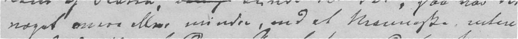noget mere eller mindre, end et Menneske, enten
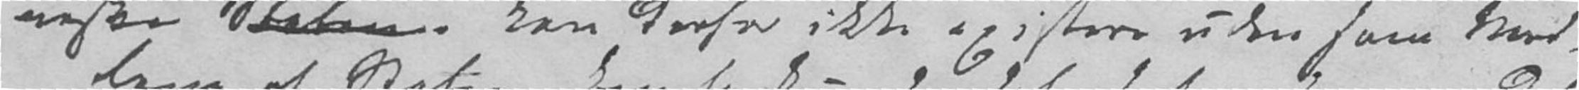neske Staten kan derfor ikke existere uden som Med-
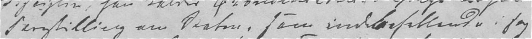Forestilling om Staten, som indbefattende i sig
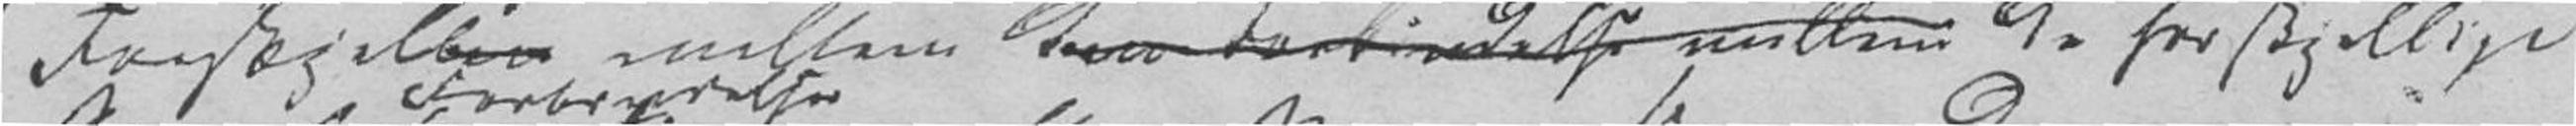Forskjeller mellem den Forbindelse mellem de forskjellige
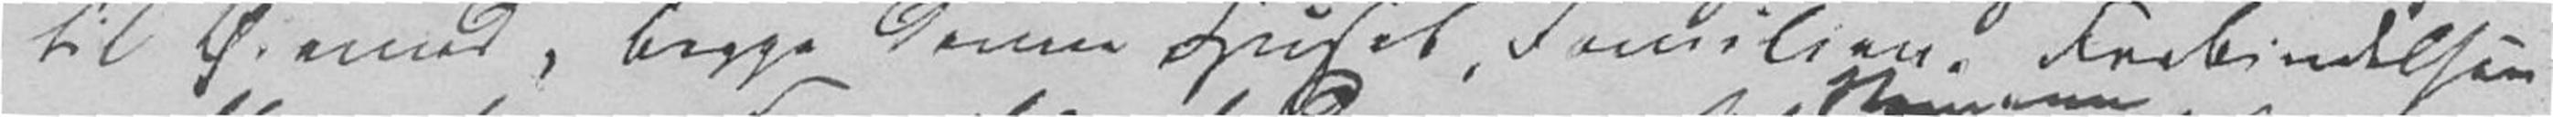til Øiemed, begge denne Huset, Familien. Forbindelsen
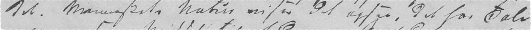det. Menneskets Natur viser det ogsaa, det har Tale-
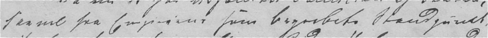saavel fra Empiriens som Begrebets Standpunct,
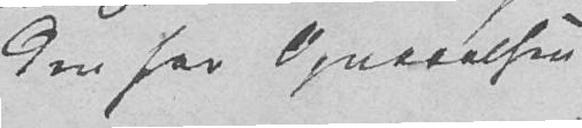der har Opnaaelsen
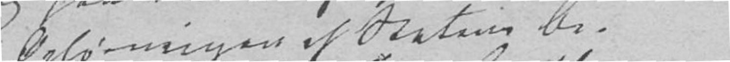Opløsningen af Statens Be-
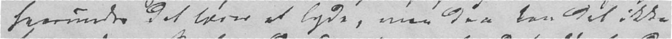hvorunder det lærer at lyde, men den kan det ikke
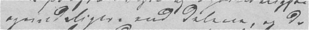oprindeligere end Delene, og de
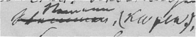X (gresk)
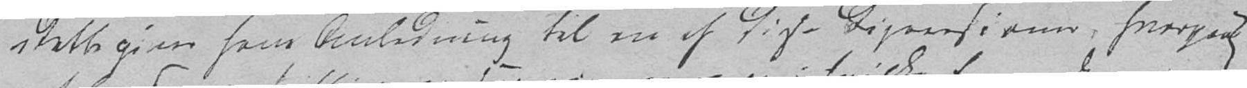Dette giver ham Anledning til en af disse Dispensioner, hvorpaa
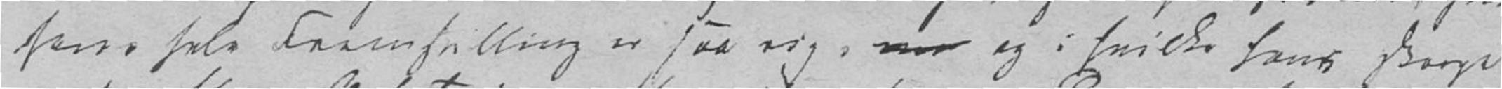hans hele Fremstilling er saa rig, men og i hvilke hans skarpe
